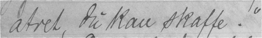atret, du kan skaffe."
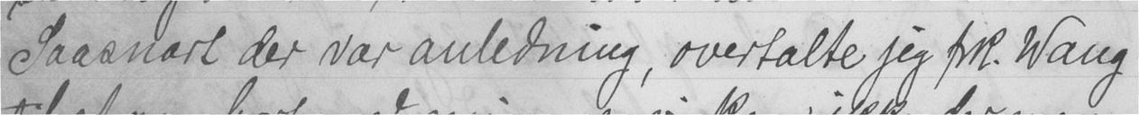Saasnart der var anledning, overtalte jeg frk. Wang
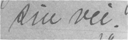sin vei.
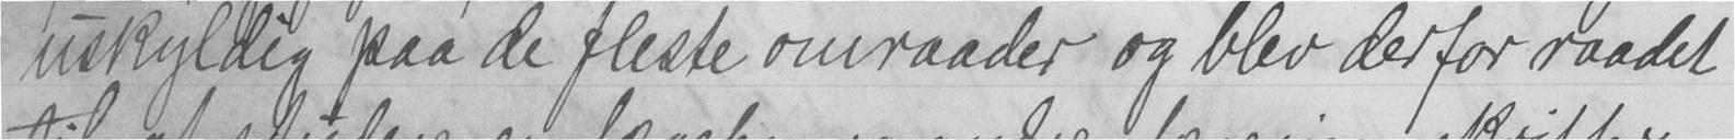uskyldig paa de fleste omraader og blev derfor raadet
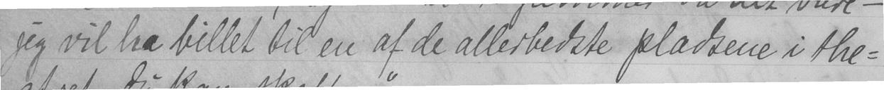jeg vil ha billet til en af de allerbedste pladsene i the-
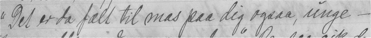"Det er da fælt til mas paa dig ogsaa, unge -
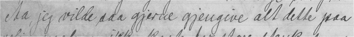Aa, jeg vilde saa gjerne gjengive alt dette paa
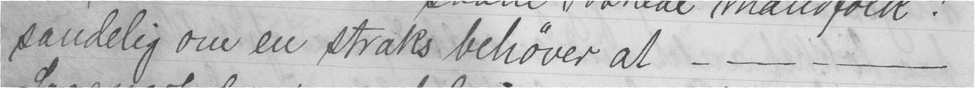sandelig om en straks behøver at - - - -
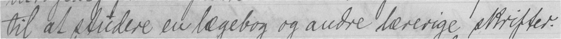til at studere en lægebog og andre lærerige skrifter.
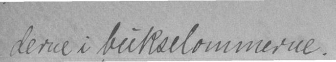derne i bukselommerne.
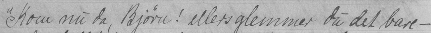"Kom nu da, Bjørn! ellers glemmer du det bare -
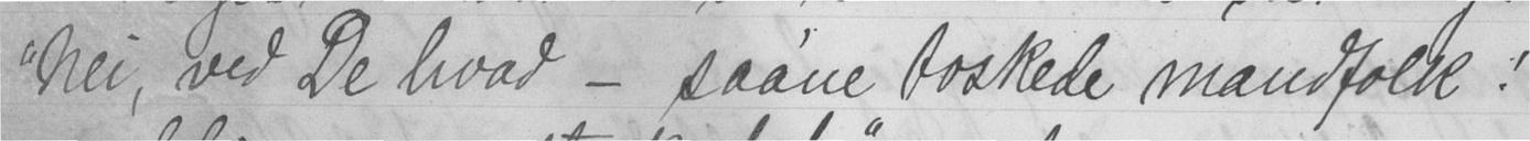"Nei, ved De hvad - saa'ne toskede mandfolk!
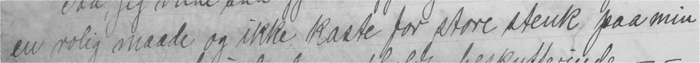en rolig maade og ikke kaste for store stenk paa min
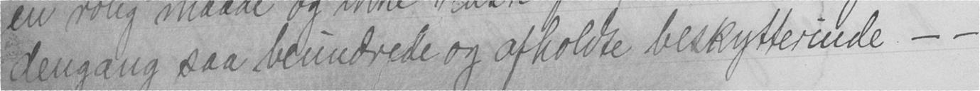dengang saa beundrede og afholdte beskytterinde - -
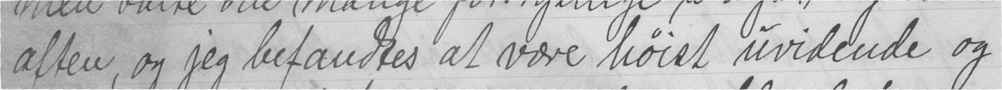aften, og jeg befandtes at være høist uvidende og
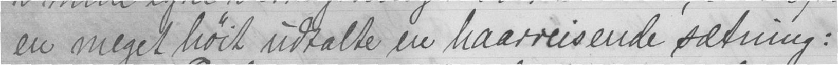en meget høit udtalte en haarreisende sætning:
